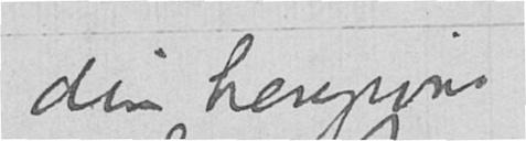din hengivne
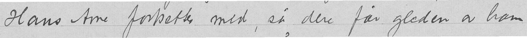Hans Arne fortsetter med, så dere får gleden av ham
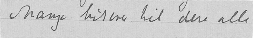Mange hilsener til dere alle
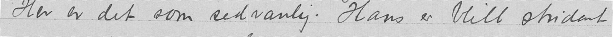Her er det som sedvanlig. Hans er blitt student
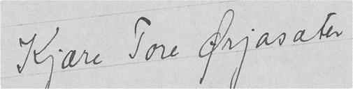Kjære Tore Ørjasæter
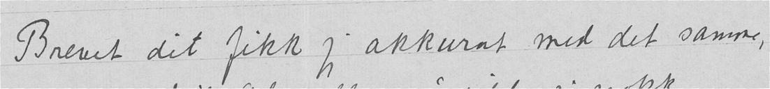Brevet dit fikk jeg akkurat med det samme,
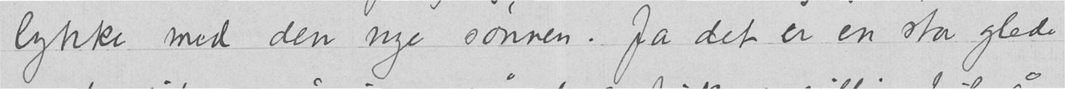lykke med den nye sønnen. Ja det er en stor glede
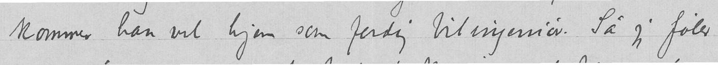kommer han vel hjem som ferdig bilingeniør. Så jeg føler
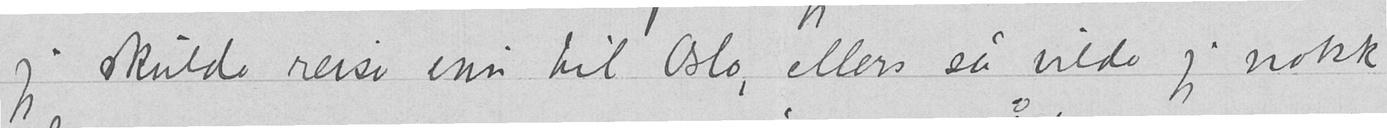jeg skulde reise inn til Oslo, ellers så vilde jeg nokk
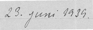23. juni 1939.
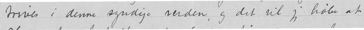trives i denne syndige verden, og det vil jeg håbe at
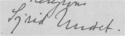Sigrid Undset.
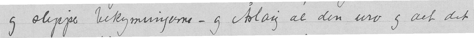og slipper bekymringerne – og Åslaug al den uro og alt det
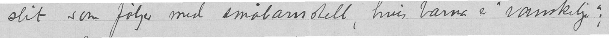slit som følger med småbarnstell, hvis barna er "vanskelige";
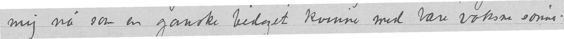mig nå som en ganske bedaget kvinne med bare voksne sønner.
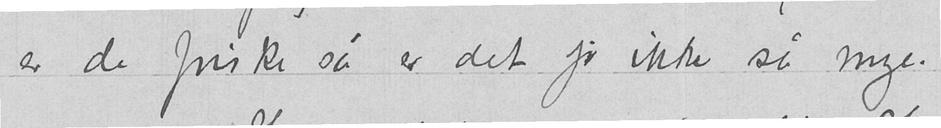er de friske så er det jo ikke så mye.
